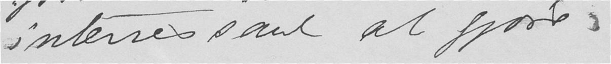interressant at gjøre.
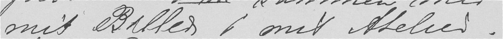mit Billed i mit Atelier.
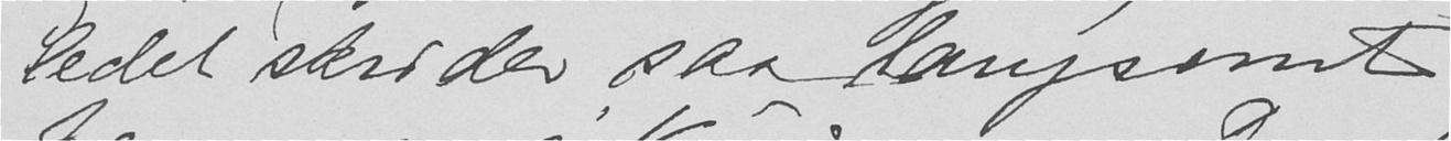ledet skrider saa langsomt
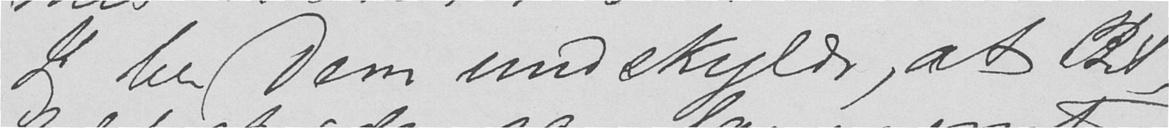Jeg ber Dem undskylde, at Bil-
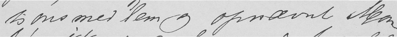tionsmedlem og opnævnt Mon-
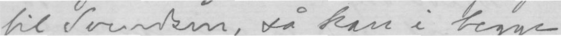til Svendsen, så kan i begge
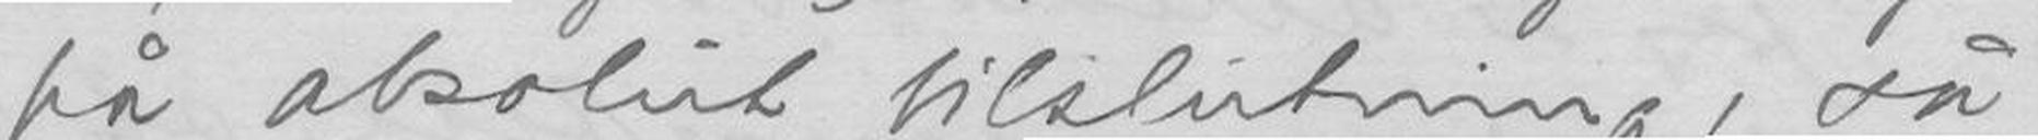på absolut tilslutning, så
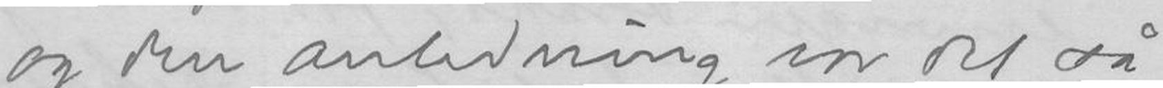og den anledning var det så
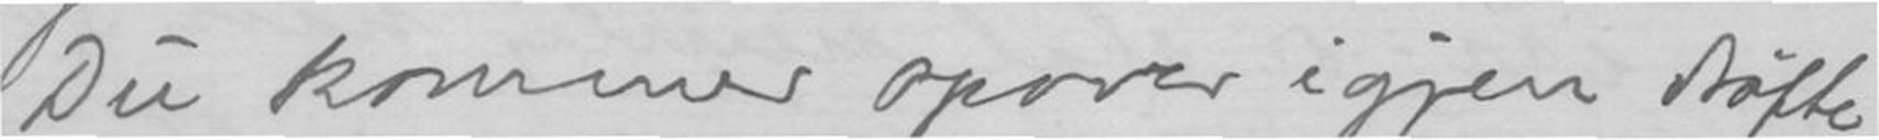Du kommer opover igjen drøfte
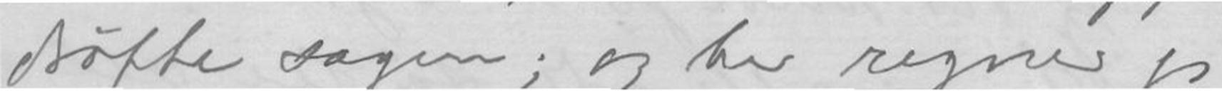drøfte sagen; og her regner jeg
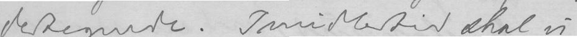dertegnede. Imidlertid skal vi
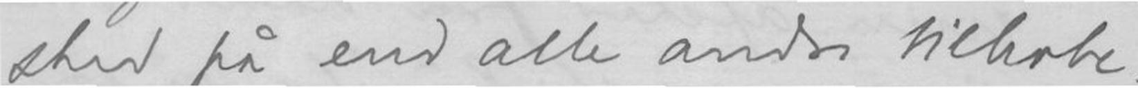sked på end alle andre tilhobe
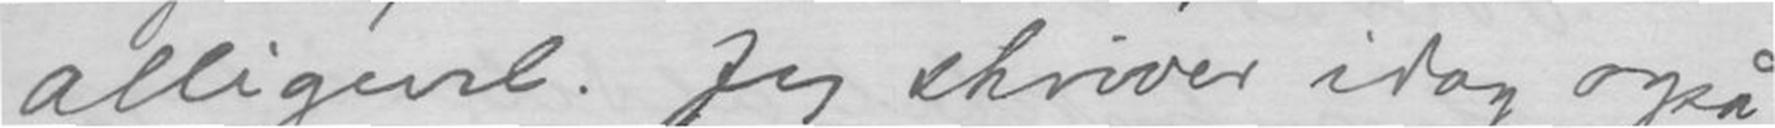alligevel. Jeg skriver idag også
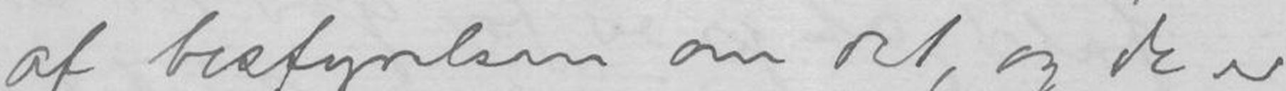af bestyrelsen om det, og de er
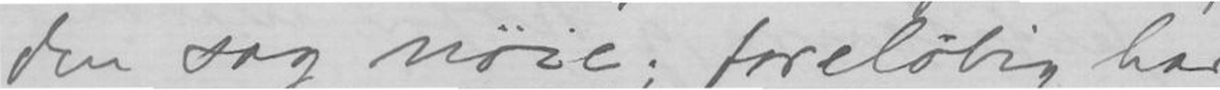den sag nøie; foreløbig har
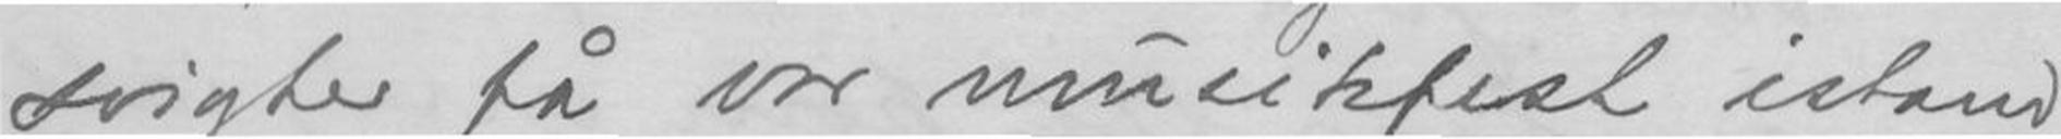svigter få vor musikfest istand
